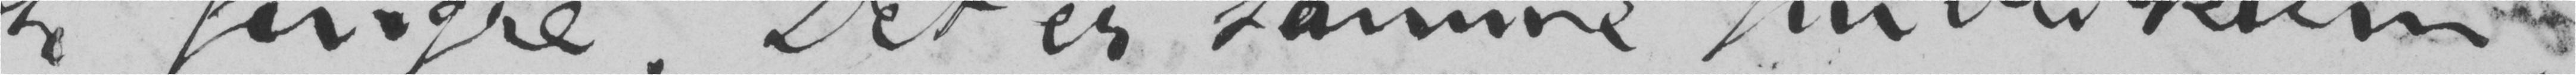fingre. Det er samme publikum,
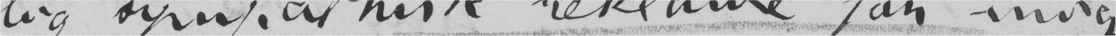lig sympathisk reklame for mig.
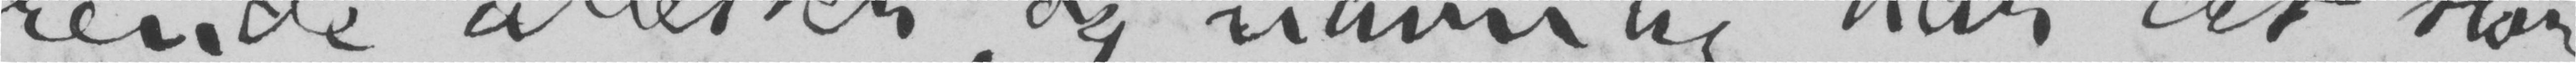rende attester, og navnlig har det store
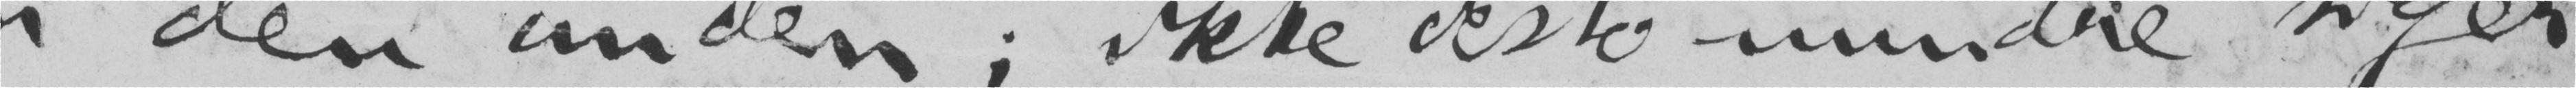den anden; ikke desto mindre siger
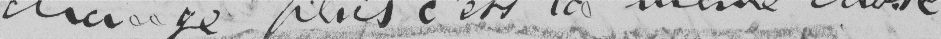change, plus c’est la même chose.
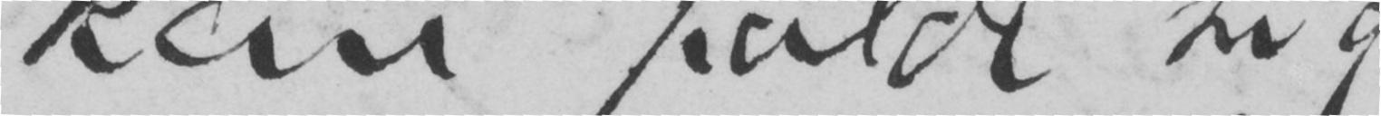kan falde sig.
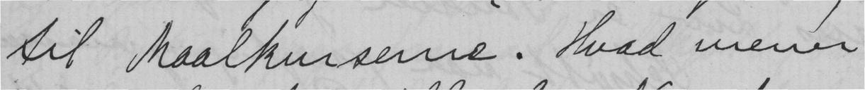til maalkurserne. Hvad mener
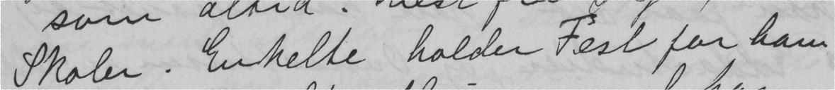Skoler. Enkelte holder Fest for ham
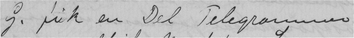G. fik en Del Telegrammer
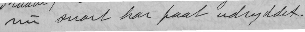nu snart har faat udryddet.
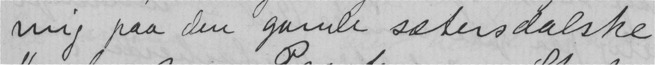mig paa den gamle sætersdalske
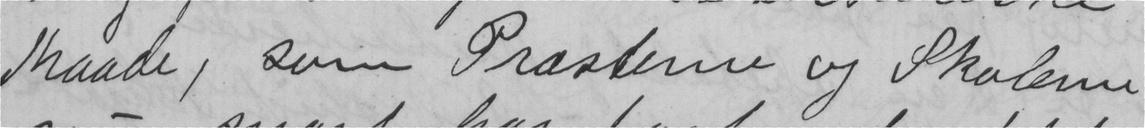Maade, som Præsterne og Skolerne
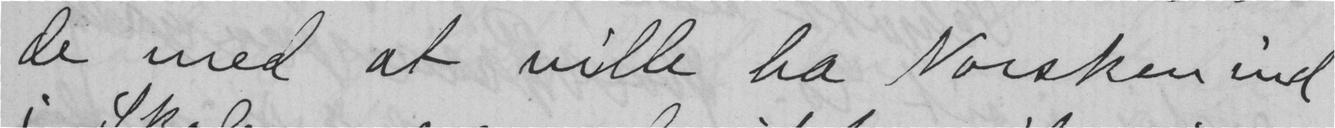de med at ville ha Norsken ind
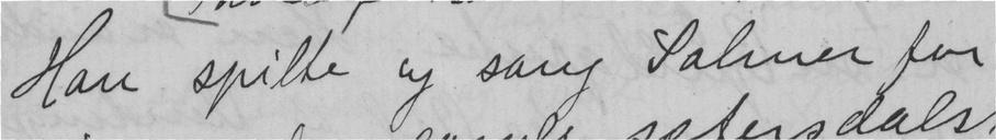Han spilte og sang Salmer for
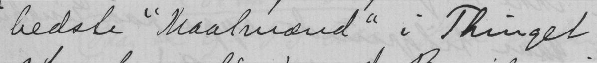bedste "Maalmænd" i Thinget
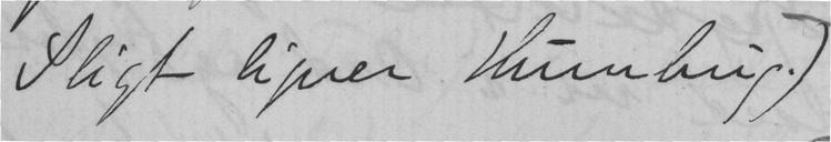Sligt ligner Humbug.)
